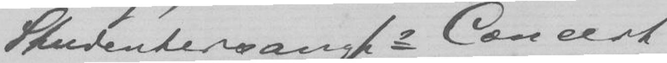Studentersangfs Concert
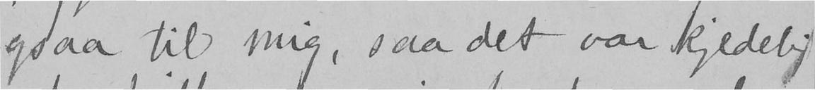ogsaa til mig, saa det var kjedelig
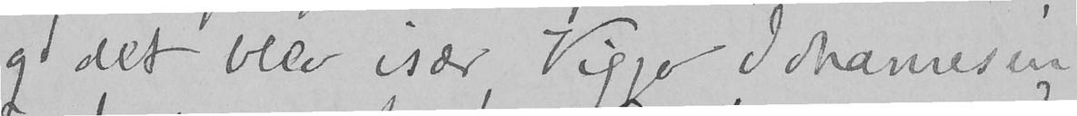og det blev især Viggo Johannesen
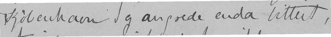Kjøbenhavn og angrede enda bittert,
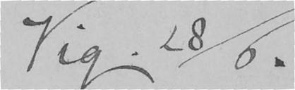Vig. 28/6.
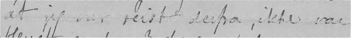at jeg var reist derfra, ikke var
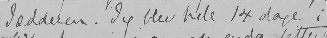Jædderen. Jeg blev hele 14 dage i
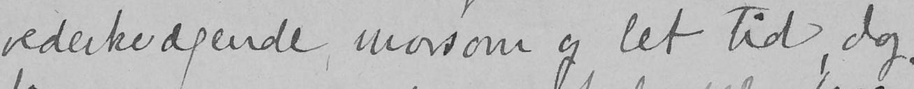vederkvægende, morsom og let tid, dag-
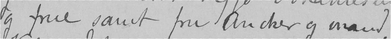og frue samt fru Ancker og mand,
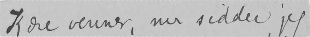Kjære venner, nu sidder jeg
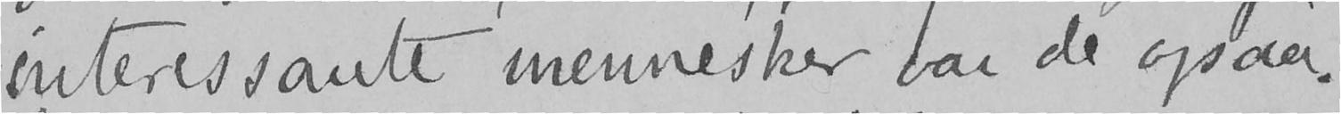interessante mennesker var de ogsaa.
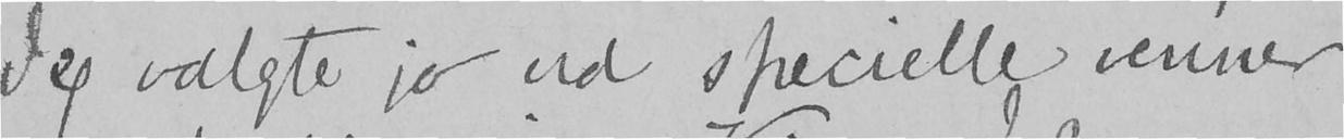Jeg valgte jo ud specielle venner,
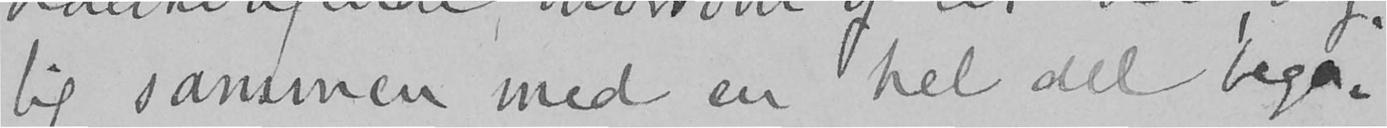lig sammen med en hel del bega-
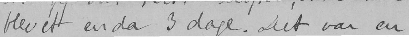blevet enda 3 dage. Det var en
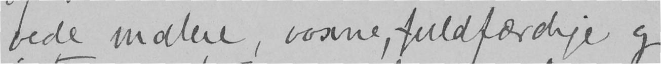vede malere, voxne, fuldfærdige og
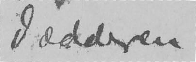Jædderen
